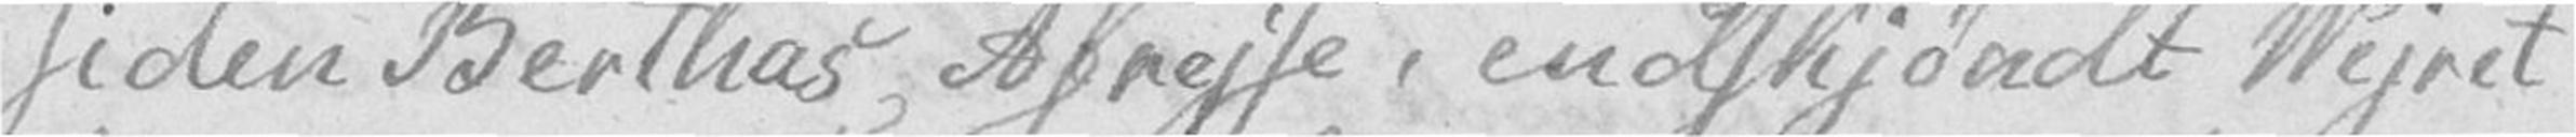siden Berthas Afrejse, endskjøndt Vejret
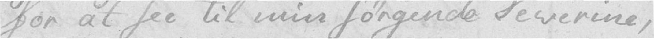for at see til min sørgende Severine,
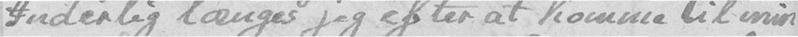Inderlig længes jeg efter at komme til min
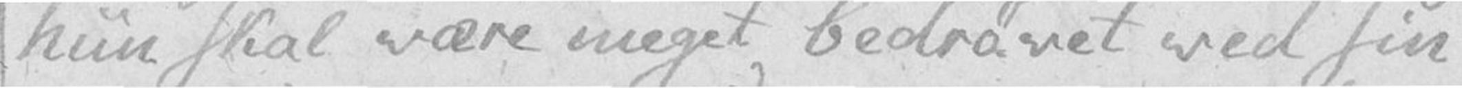hun skal være meget bedrøvet ved sin
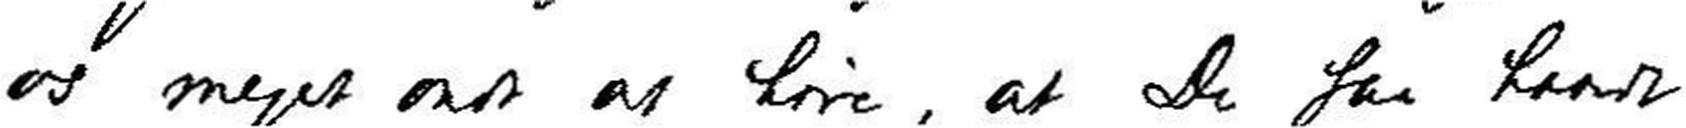os meget ondt at høre, at De saa haardt
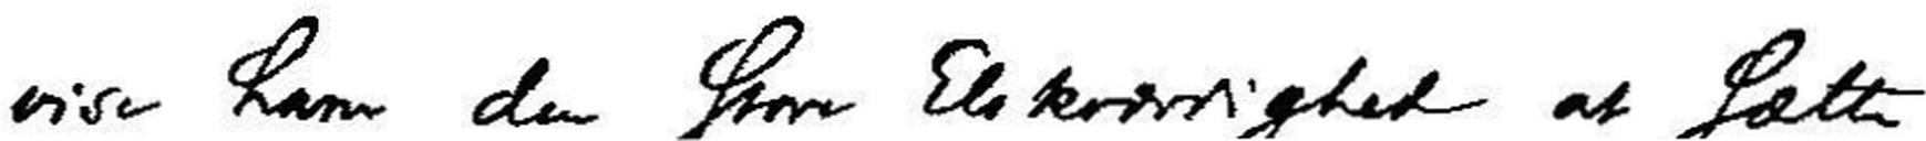vise ham den store Elskværdighed at sætte
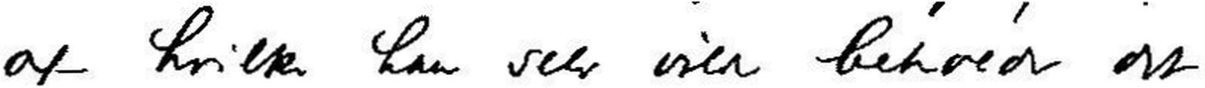af hvilke han selv vilde beholde det
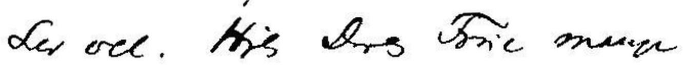Lev vel, Hils Deres Frue mange
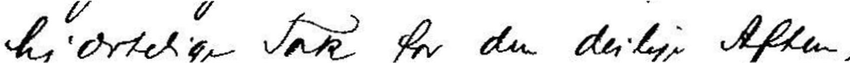hjærtelige Tak for den deilige Aften
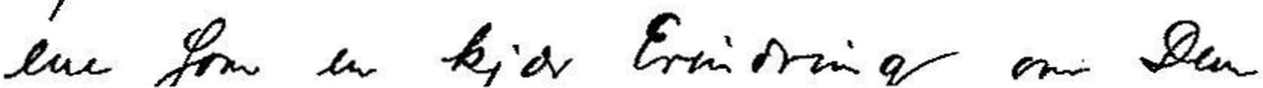ene som en kjær Erindring om Dem
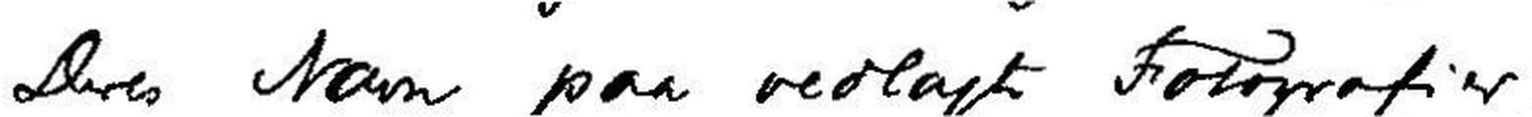Deres Navn paa vedlagte Fotografier
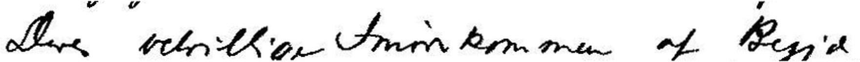Deres velvillige Imødekommen af Begjæ
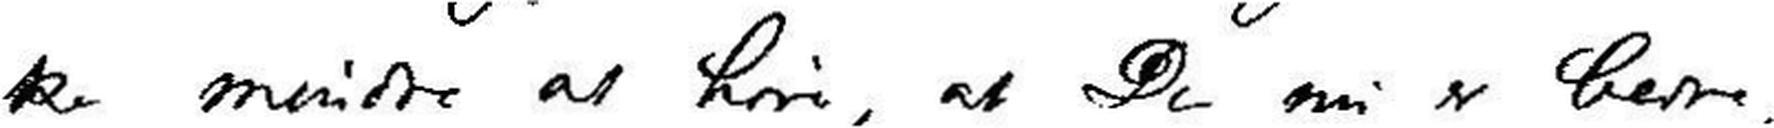ke mindst at høre, at De nu er bedre.
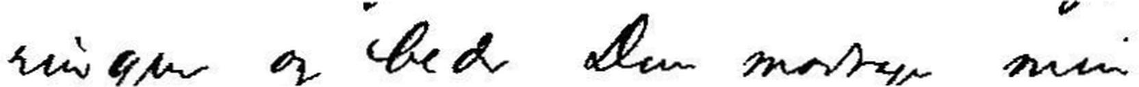ringen og bed Dem modtage min
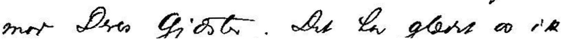mod Deres Gjæster. Det har gledet os ik
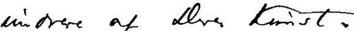undrere af Deres Kunst.
